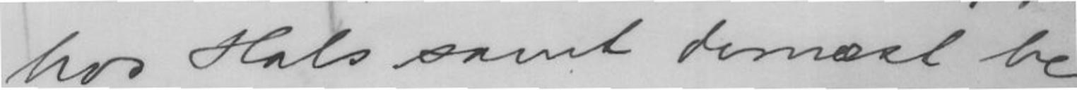hos Hals samt dernæst be-
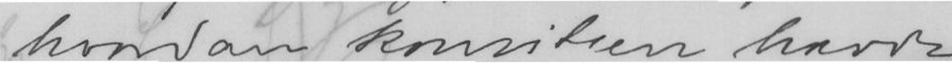hvordan komiteen havde
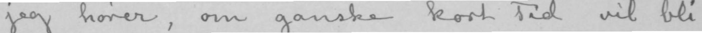jeg hører, om ganske kort Tid vil bli-
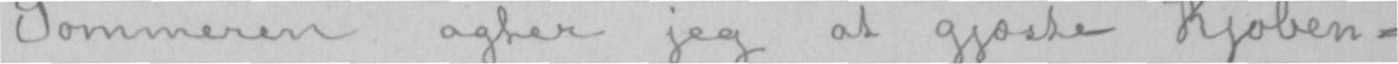Sommeren agter jeg at gjæste Kjøben-
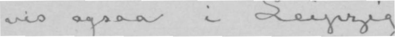vis ogsaa i Leipzig.
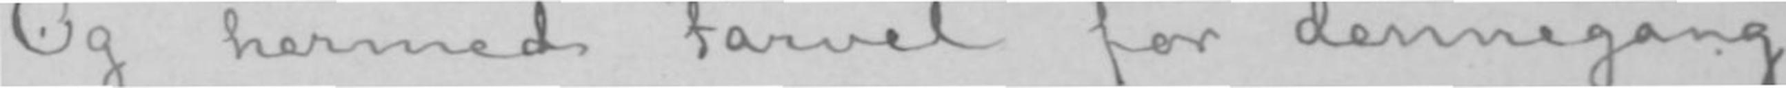Og hermed Farvel for dennegang.
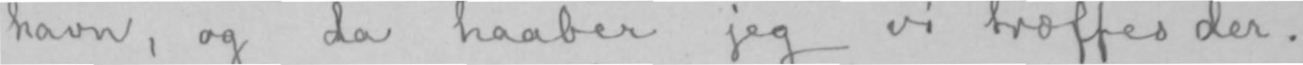havn, og da haaber jeg vi træffes der.
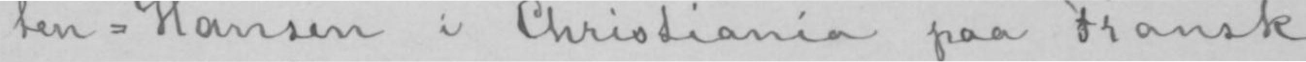ten-Hansen i Christiania paa Fransk
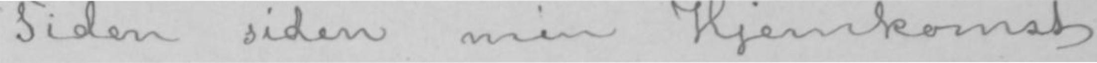Tiden siden min Hjemkomst
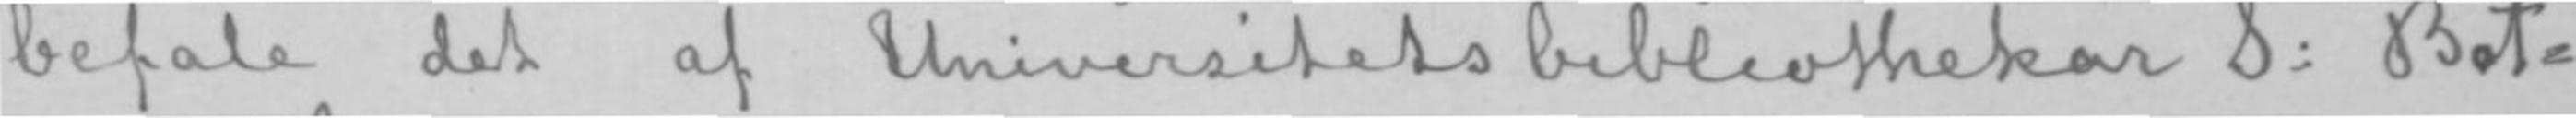befale det af Universitetsbibliothekar P: Bot-
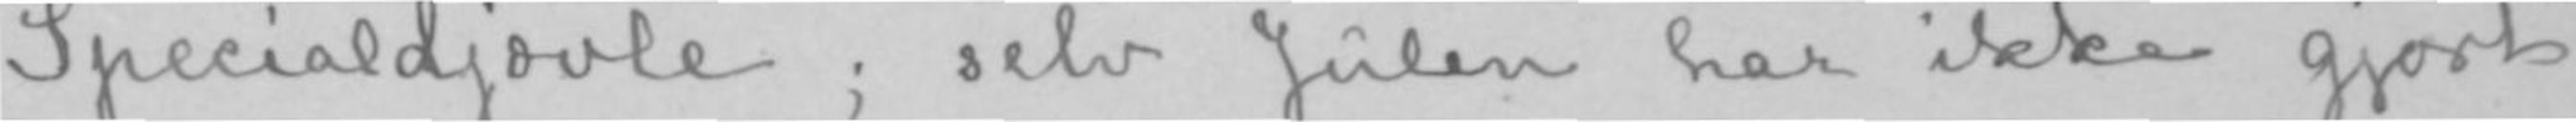Specialdjævle; selv Julen har ikke gjort
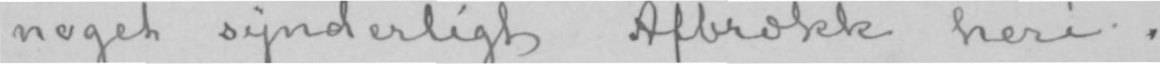noget synderligt Afbrækk heri.
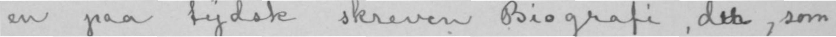en paa tydsk skreven Biografi, derd, som
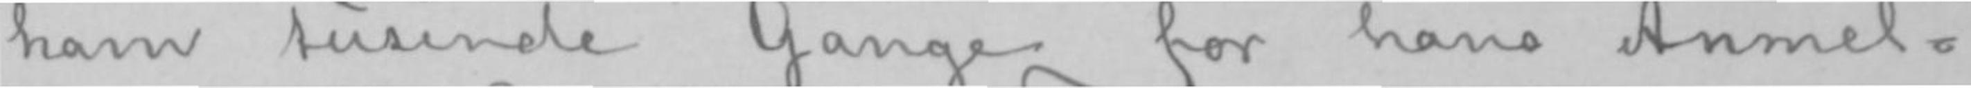ham tusinde Gange for hans Anmel-
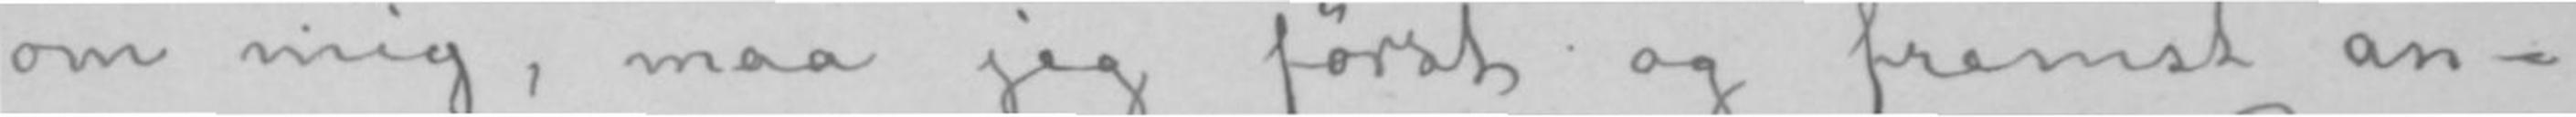om mig, maa jeg først og fremst an-
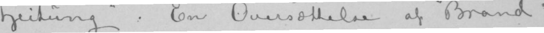Zeitung». En Oversættelse af «Brand»
